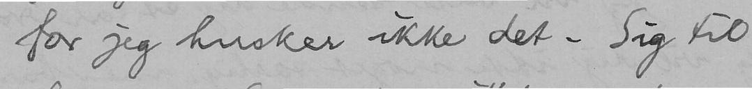for jeg husker ikke det. Sig til
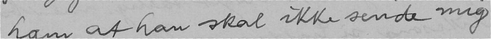ham at han skal ikke sende mig
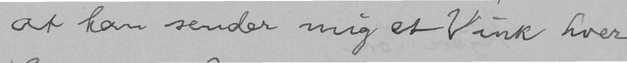at han sender mig et Vink hver
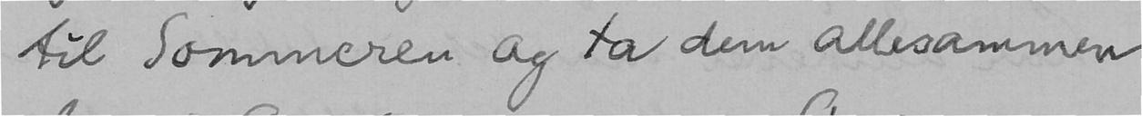til Sommeren og ta dem allesammen
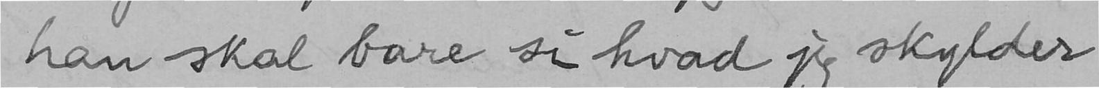han skal bare si hvad jeg skylder
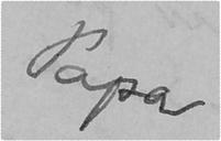Papa
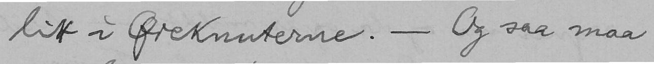litt i Øreknuterne. - Og saa maa
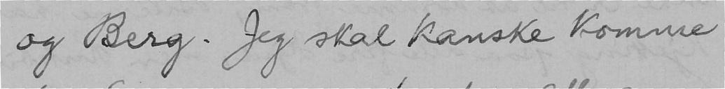og Berg. Jeg skal kanske komme
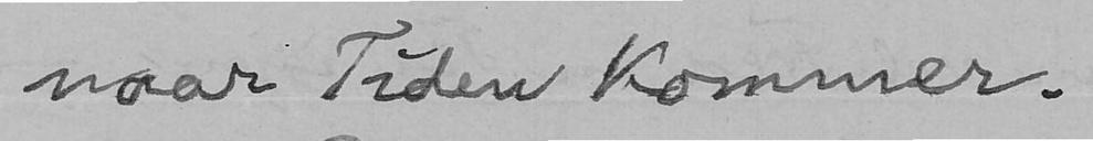naar Tiden kommer.
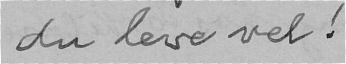du leve vel!
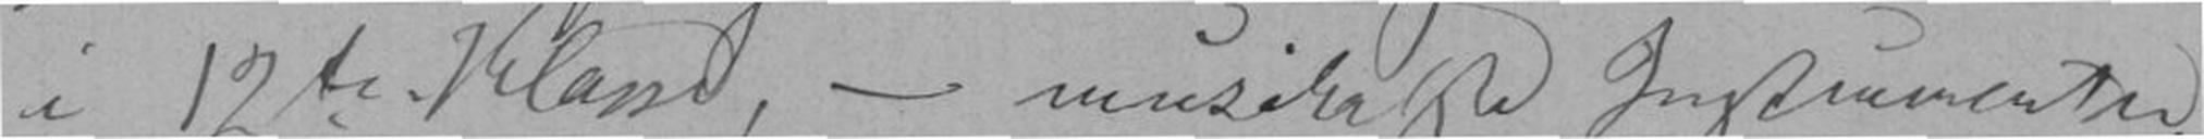i 12te Klasse, - musikalske Instrumenter,
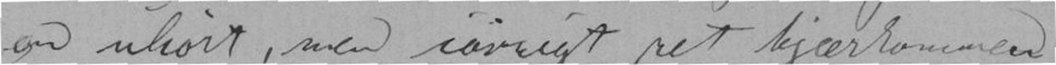en uhørt, men iøvrigt ret kjærkommen
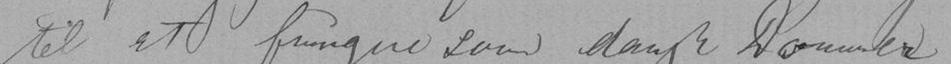til at fungere som dansk Dommer
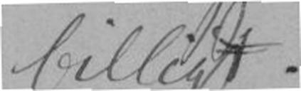billigt.
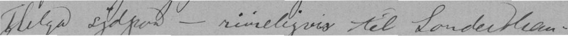Helga sydpaa - rimeligvis til Sondershau-
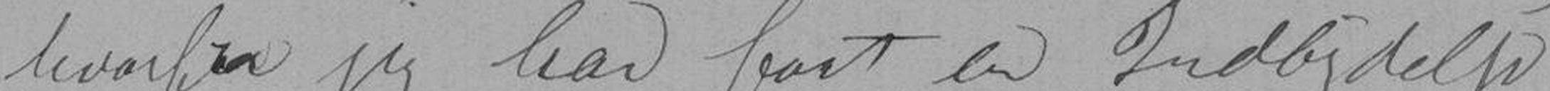hvorfra jeg har faat en Indbydelse
